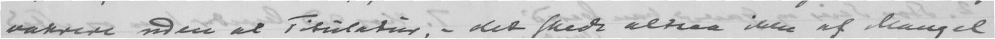vakrere uden al Titulatur, - det skede altsaa ikke af Mangel
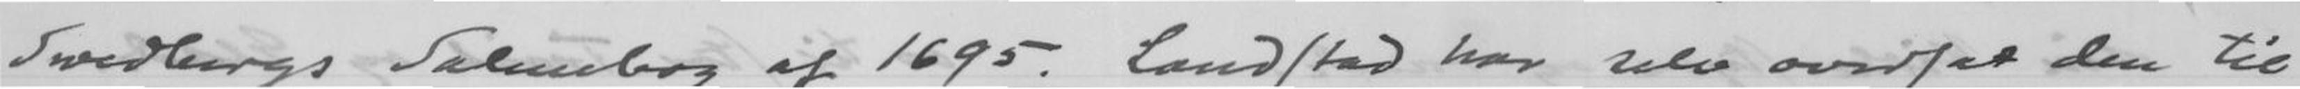Swedbergs Salmebog af 1695. Landstad har selv oversat den til
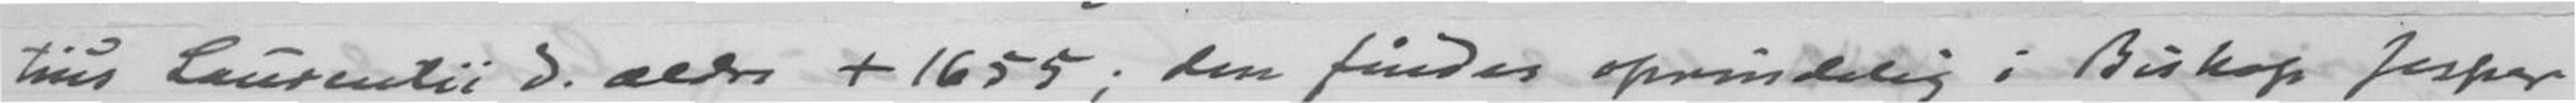tius Lauren tii d. ældre + 1655; den findes oprindelig i Biskop Jesper
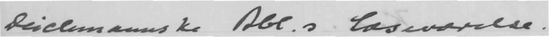Disckmannske Abl.s Læseværelse.
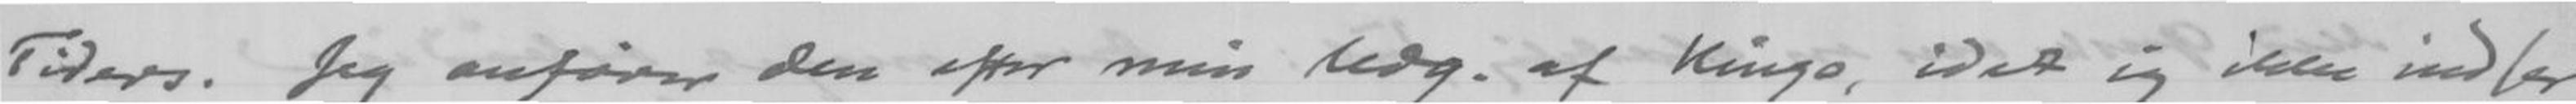Tiders. Jeg anfører den efter min Udg. af Kingo, idet jeg ikke indser
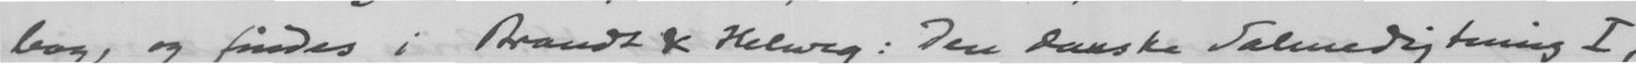bog, og findes i Brandt & Helweg: Den danske Salmedigtning I,
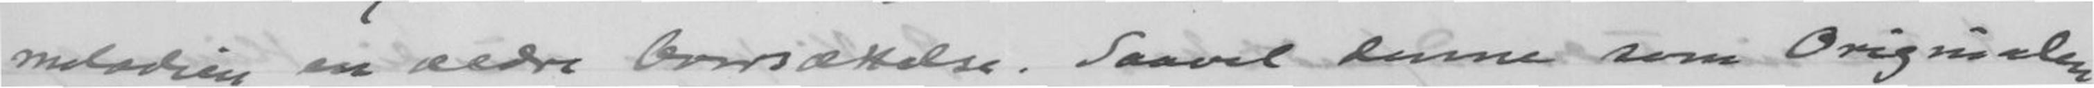melodien en ældre Oversættelse. Saavel denne som Originalen
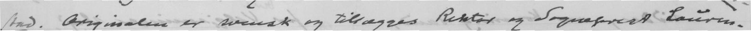stad. Originalen er svensk og tillægges Rektor og Sogneprest Lauren-
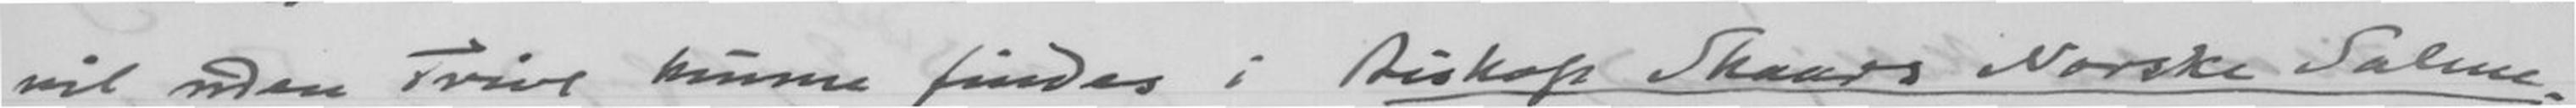vil uden Tvivl kunne findes i Biskop Skaars Norske Salme-
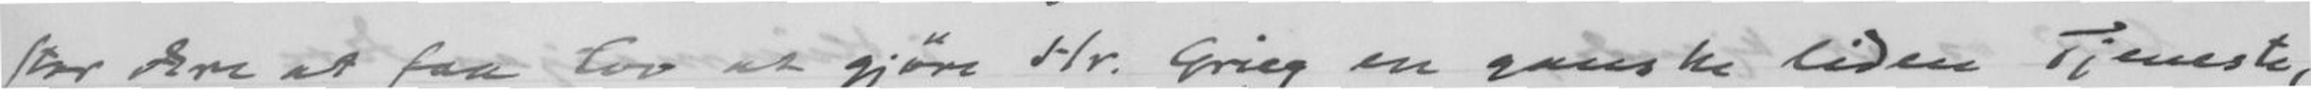Stor Ære at faa Lov at gjøre Hr. Grieg en ganske liden Tjeneste,
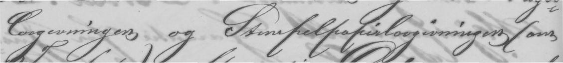lovgivningen og Stempelpapirlovgivingen (om
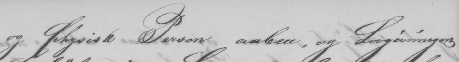og fhysisk Person aaben, og Lovgivningen
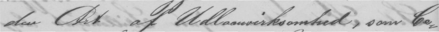den Art af Udlaanvirksomhed, som be-
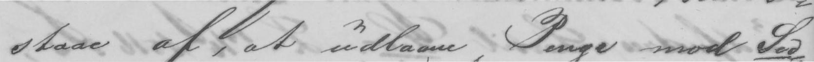staae af, at udlaane Penge mod Sed-
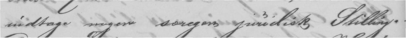indtage nogen særegen juridisk Stilling. -
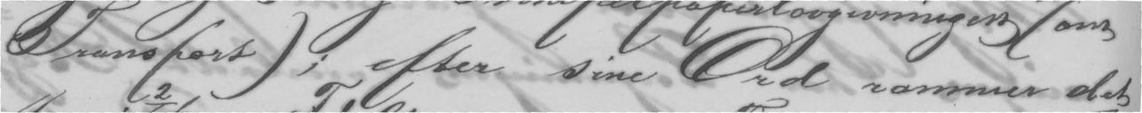Transport); efter sine Ord rammer det-
